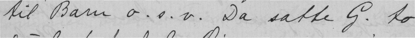til Barn o. s.v. Da satte G. to
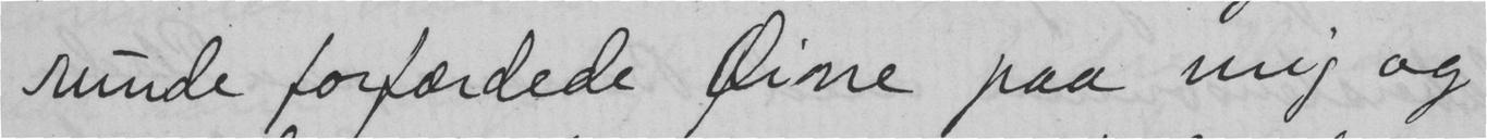runde forfærdede Øine paa mig og
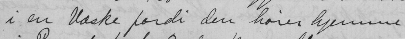i en Væske fordi den hører hjemme
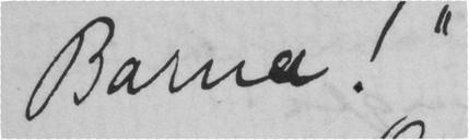Barna!"
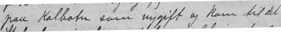paa Kolbotn som nygift og kom til det
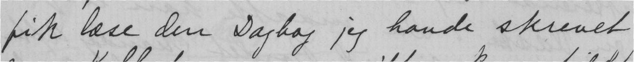fik læse den Dagbog jeg havde skrevet
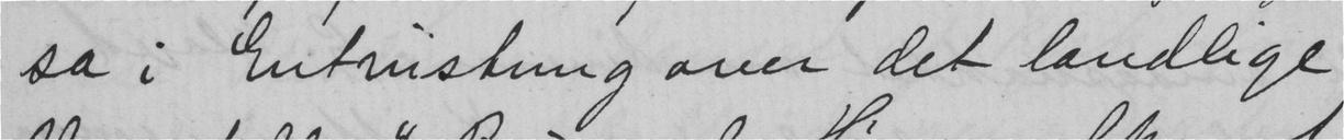sa i Entristung over det landlige
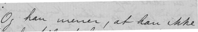Og han mener, at han ikke
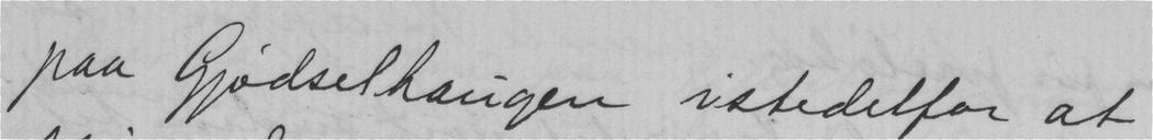paa Gjødselhaugen istedetfor at
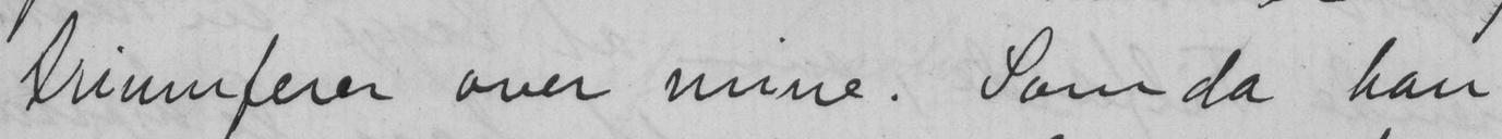trimferer over mine. Som da han
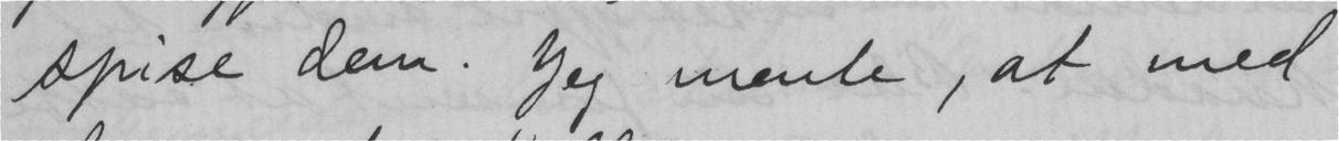spise dem. Jeg mente, at med
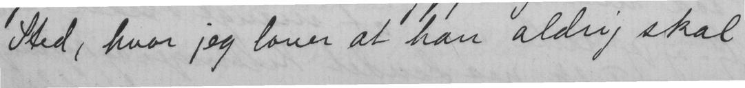Sted, hvor jeg lover at han aldrig skal
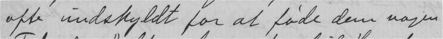ofte undskyldt for at føde dem nogen
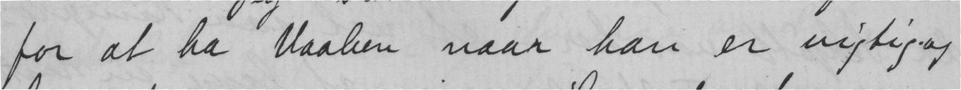for at ha Vaaben naar han er vigtig og
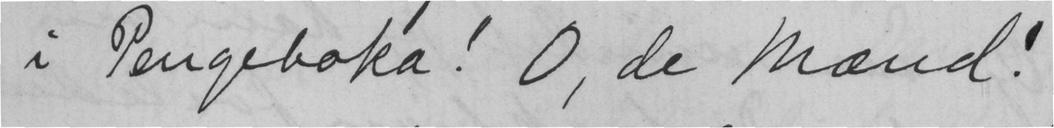i Pengeboka! O, de mænd!
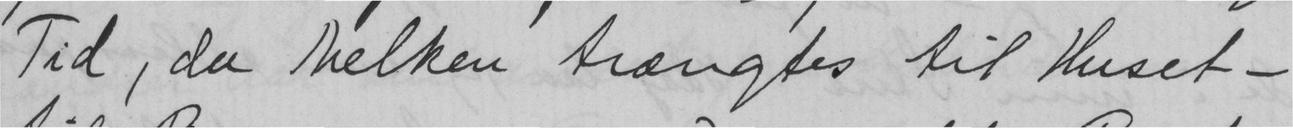Tid, da Melken trængtes til Huset -
